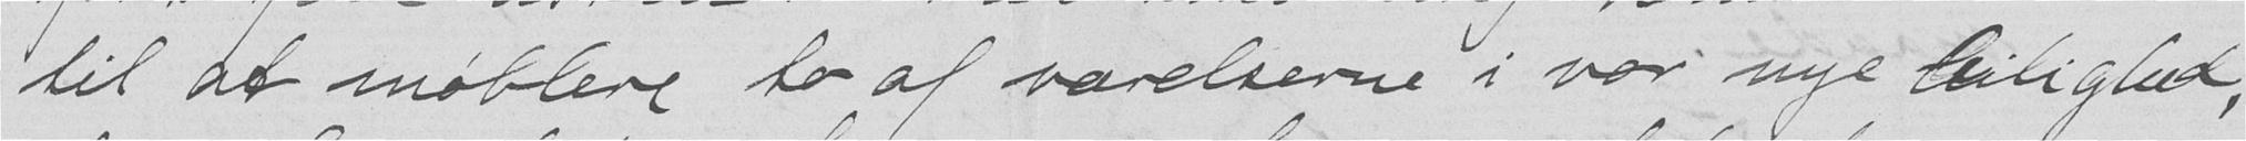til at møblere to af værelserne i vor nye leilighed,
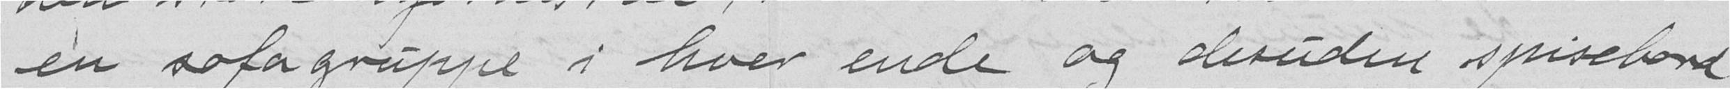en sofagruppe i hver ende og desuden spisebord
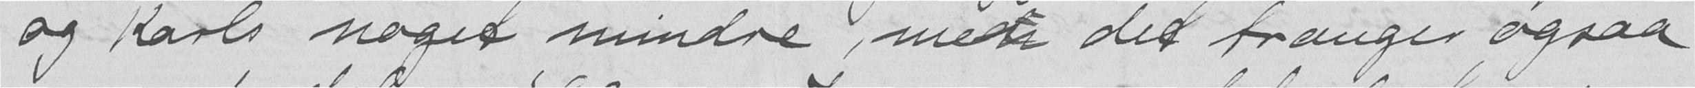og Karls noget mindre, men det trænger ogsaa
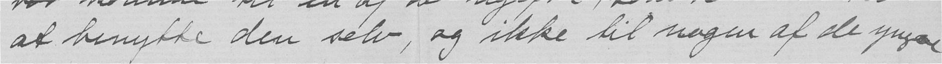at benytte den selv, og ikke til nogen af de yngre
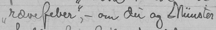"rævefeber", - om du og Münster
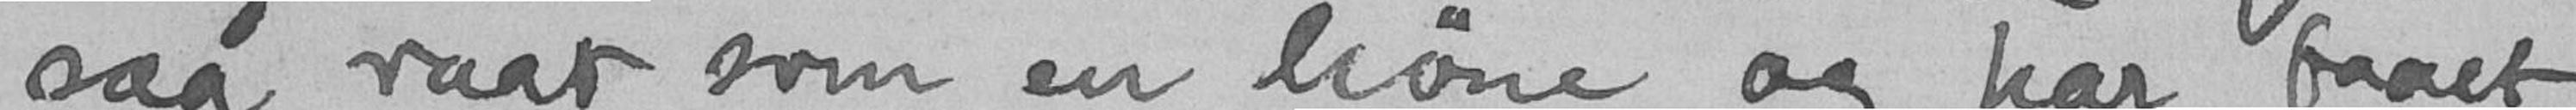saa vaat som en høne og har faaet
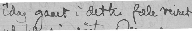idag gaaet i dette fæle veiret
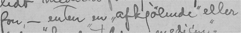fon, - enten en "afkjølende" eller
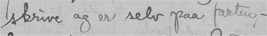skrive og er selv paa farten, -
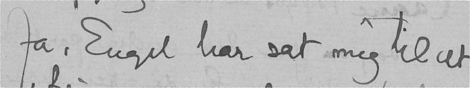Ja, Engel har sat mig til at
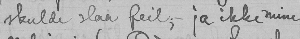skulde slaa feil; - ja ikke mine
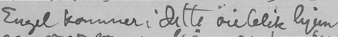Engel kommer i dette øieblik hjem
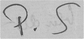P. S
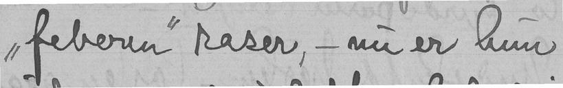"feberen" raser, - nu er hun
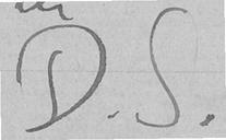D. S.
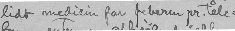lidt medicin for feberen pr. tele-
